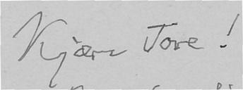Kjære Tore!
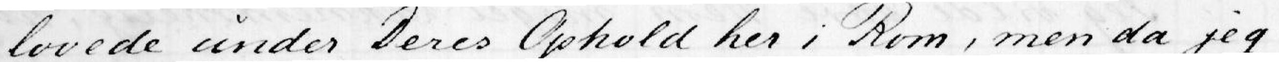lovede under Deres Ophold her i Rom, men da jeg
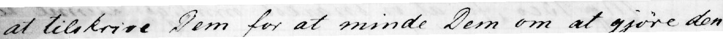at tilskrive Dem for at minde Dem om at gjøre den
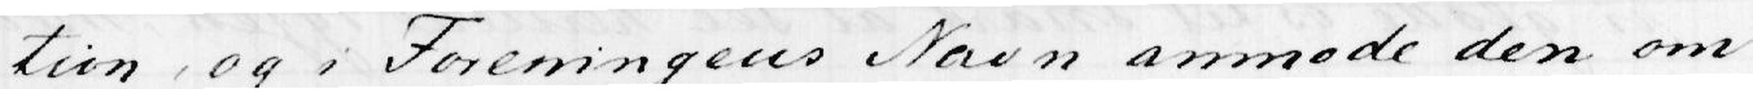tion, og i Foreningens Navn anmode den om
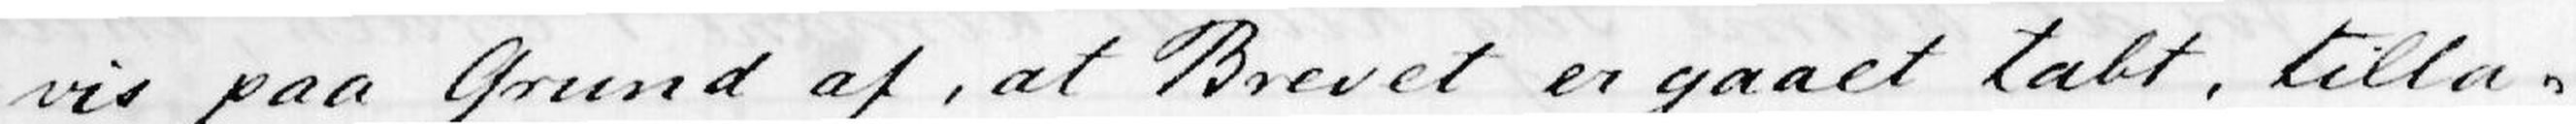vis paa Grund af, at Brevet er gaaet tabt, tilla-
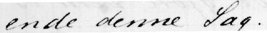ende denne Sag.
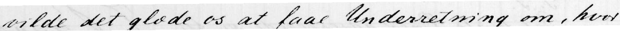vilde det glæde os at faae Underretning om, hvor
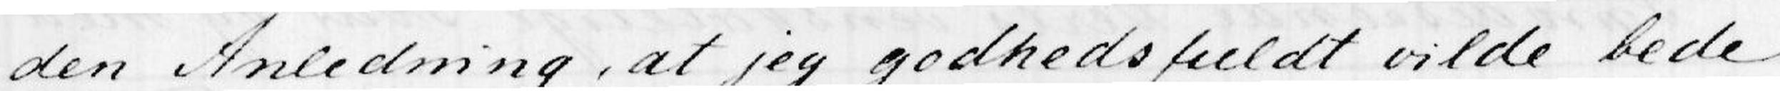den Anledning, at jeg godhedsfuldt vilde bede
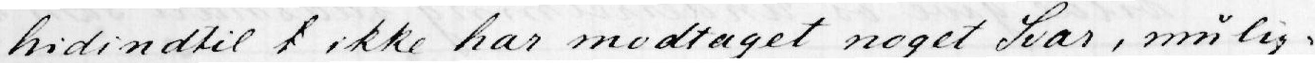hidindtil xxx ikke har modtaget noget Svar, mulig-
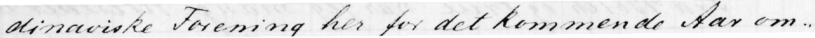dinaviske Forening her for det kommende Aar om-
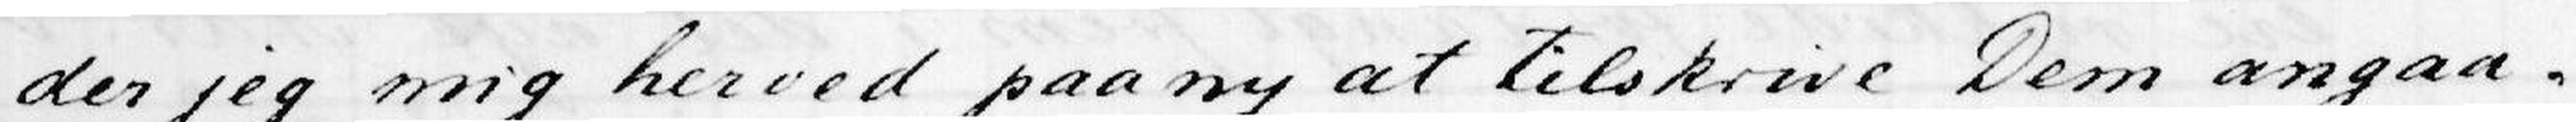der jeg mig herved påå ny at tilskrive Dem angaa-
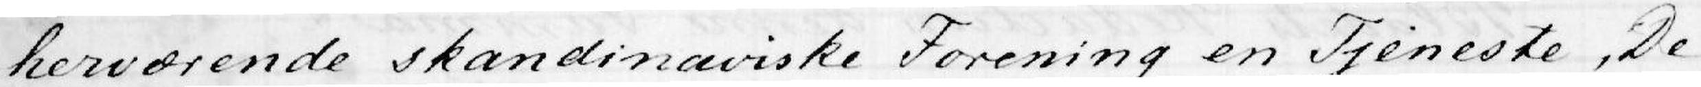herværende skandinaviske Forening en Tjeneste, De
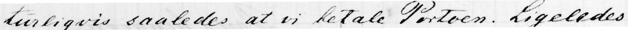turligvis saaledes at vi betale Portoen. Ligeledes
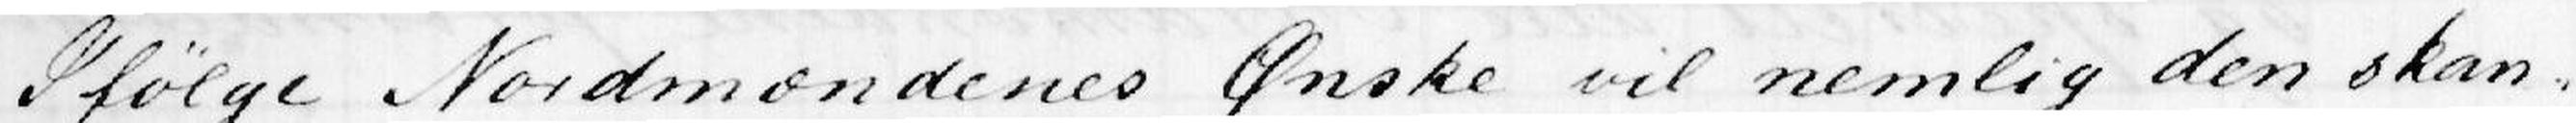Ifølge Nordmændenes Ønske vil nemlig den skan-
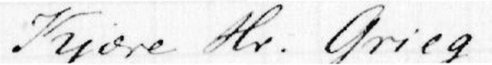Kjære Hr. Grieg!
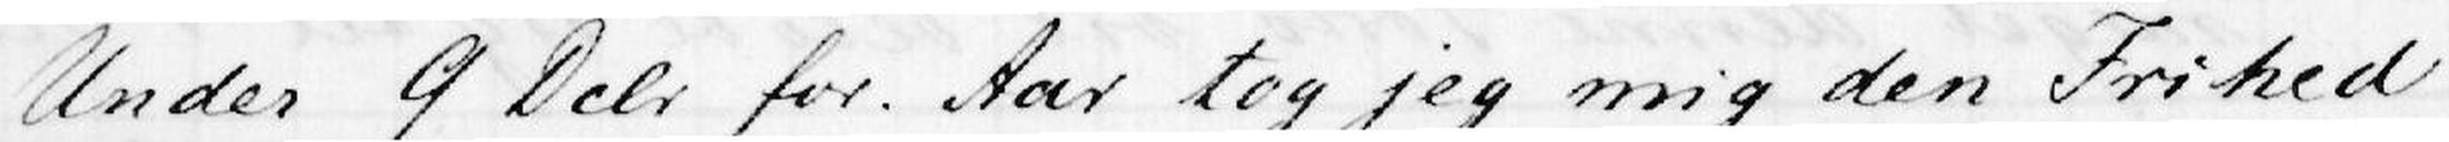Under 9 Dcbr for. Aar tog jeg mig den Frihed
